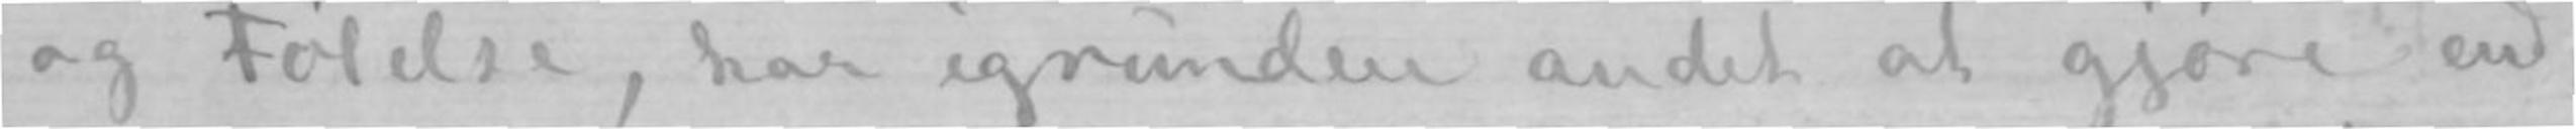og Følelse, har igrunden andet at gjøre end
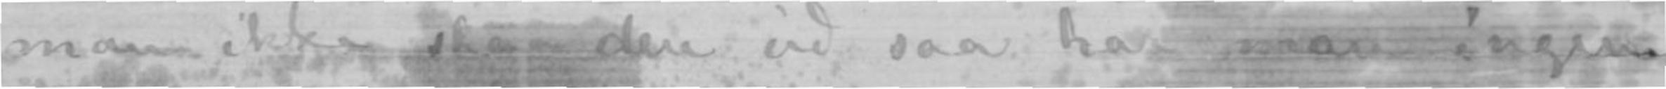man ikke staa den ud saa har man ingen
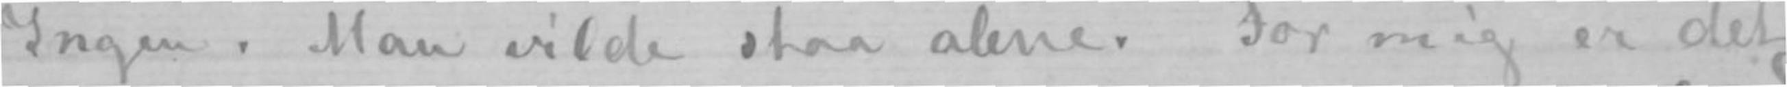Ingen. Man vilde staa alene. For mig er det
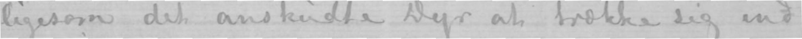ligesom det anskudte Dyr at trække sig ind
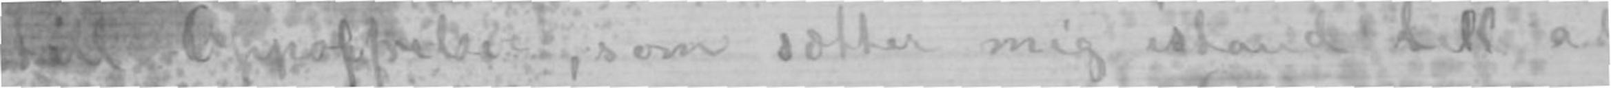till Oppoffrelser, som sætter mig istand till at
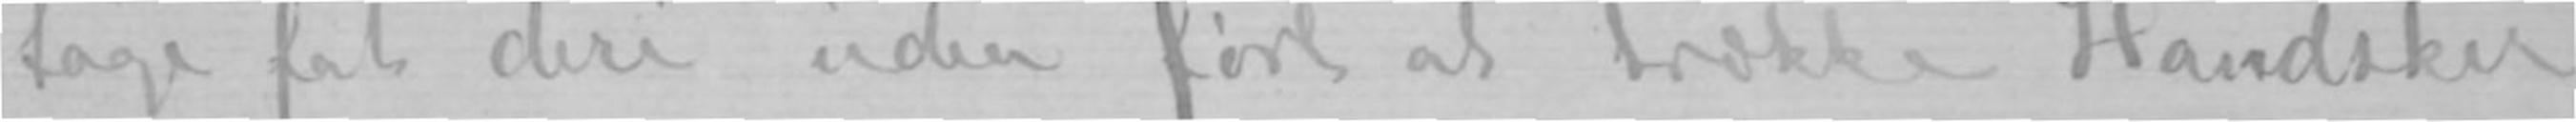tage fat deri uden ført at trække Handsker
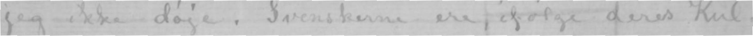jeg ikke døje. Svenskerne ere, ifølge deres Kul-
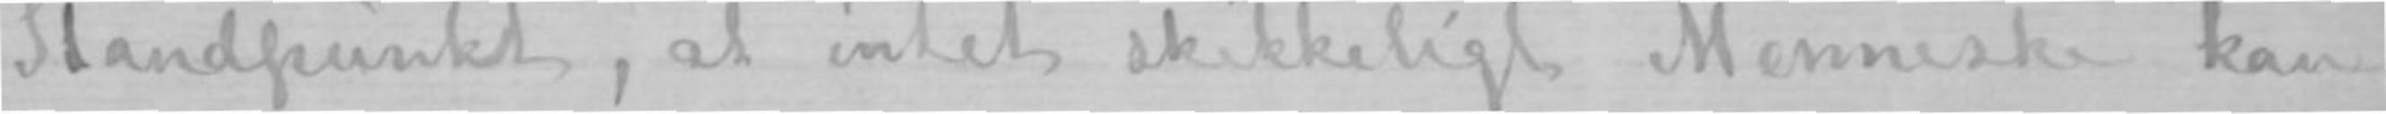Standpunkt, at intet skikkeligt Menneske kan
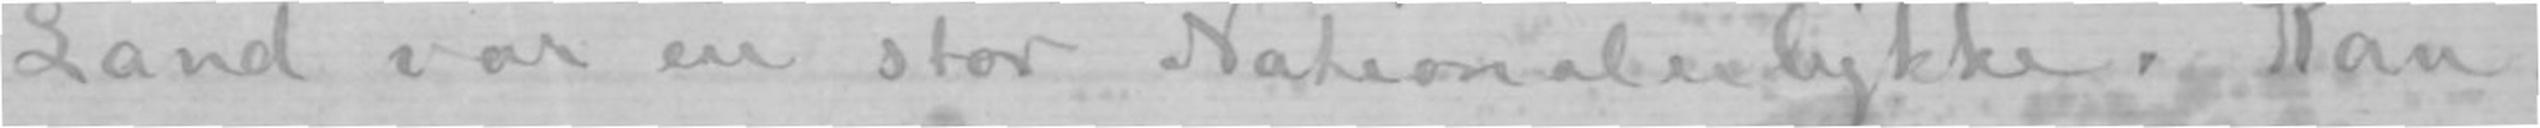Land var en stor Nationalulykke. Kan
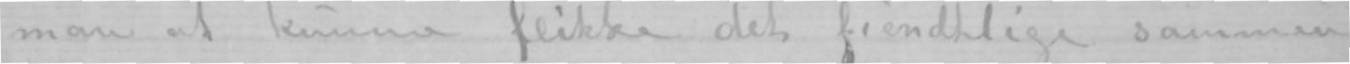man at kunne flikke det fiendtlige sammen
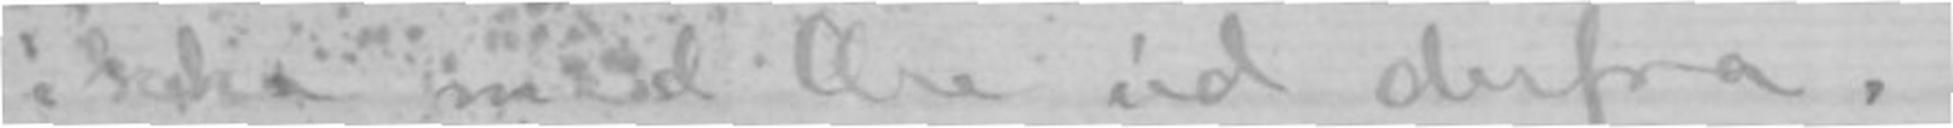ikke med Ære ud derfra.
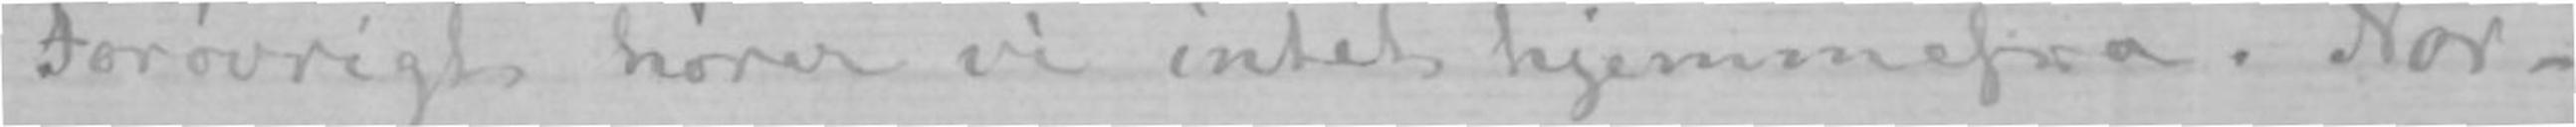Forøvrigt hører vi intet hjemmefra. Nor-
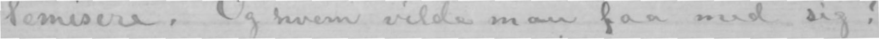lemisere. Og hvem vilde man faa med sig?
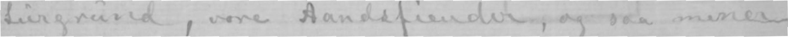turgrund, vore Aandsfiender, og saa mener
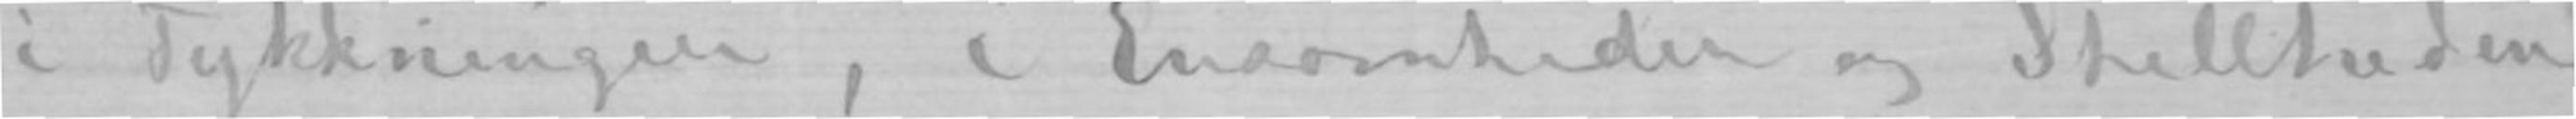i Tykkningen, i Ensomheden og Stillheden
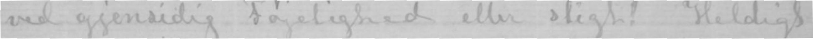ved gjensidig Føjelighed eller sligt! Heldigt
